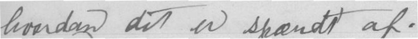hvordan det er spændt af.
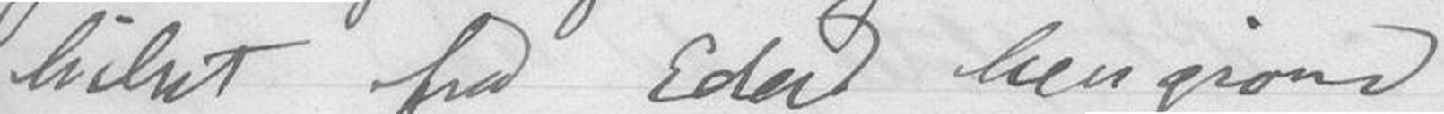hilset fra Eder hengivne
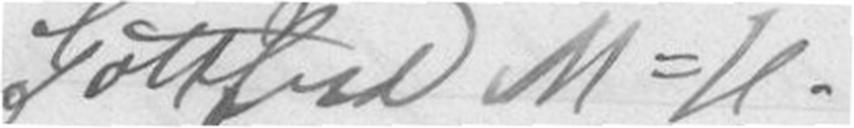Gottfred M-H.
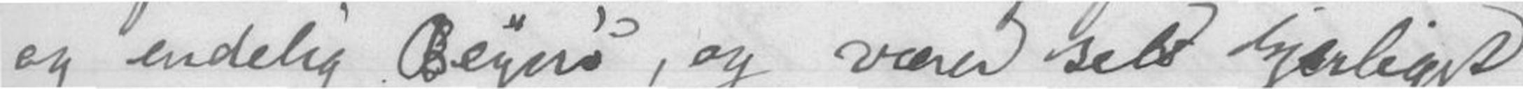og endelig Beyer's, og værer selv kjærligst
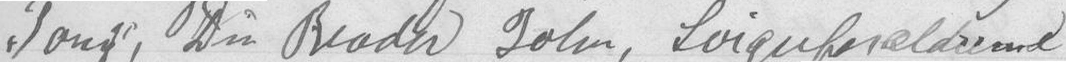Tony, Din Broder John, Svigerforældrene
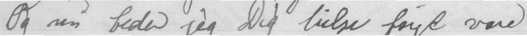Og nu beder jeg Dig hilse først vore
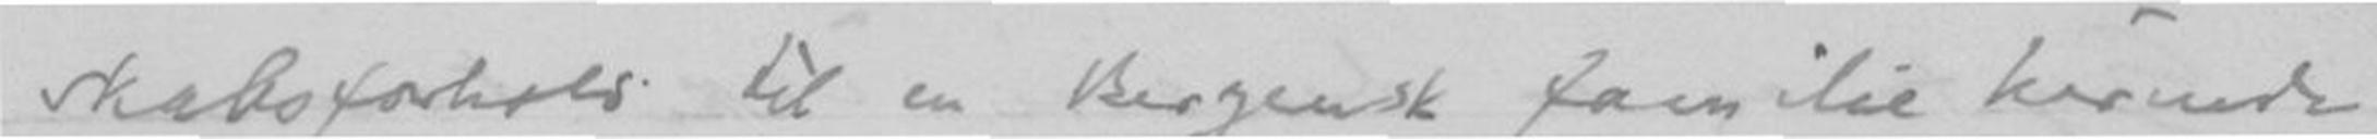skabelighed til en Bergens familie hernede
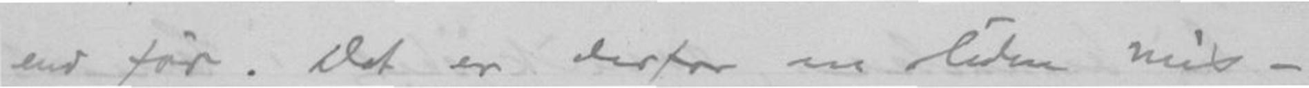end før. Det er derfor en liden mis-
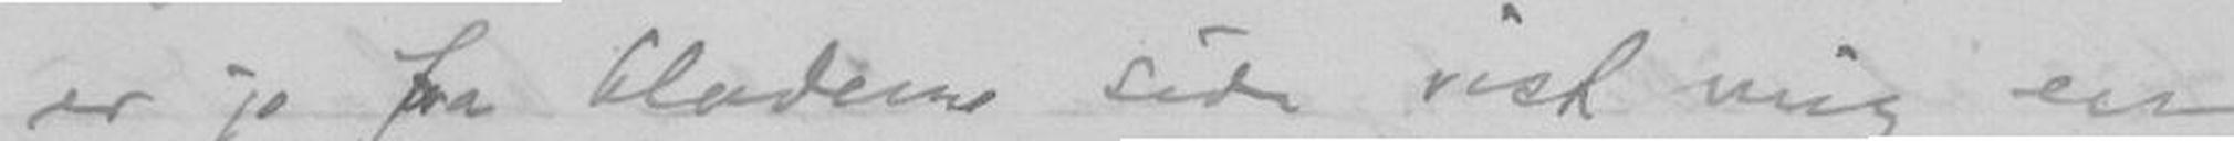er jo fra bladerne side vist mig en
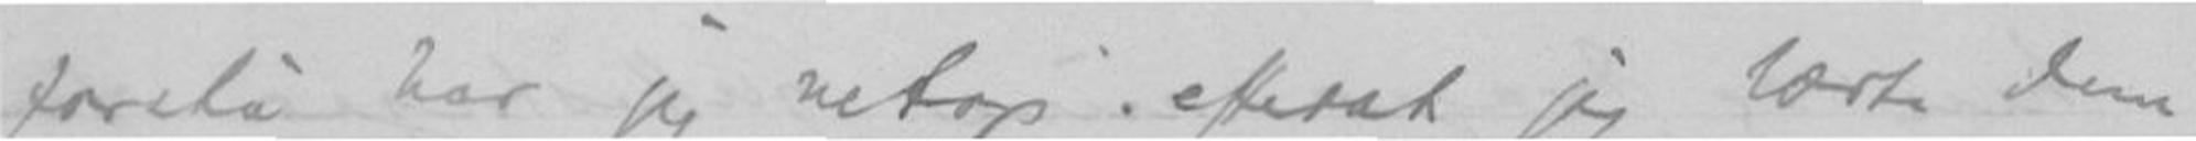forstå har jeg netop efterat jeg lærte Dem
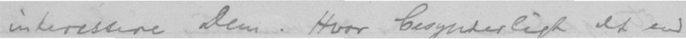interessere Dem. Hvor besynderligt det end
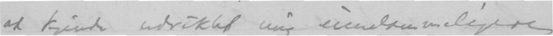at kjende udviklet mig eiendommeligere
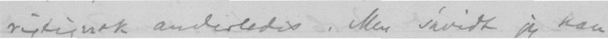rigtignok anderledes. Men såvidt jeg kan
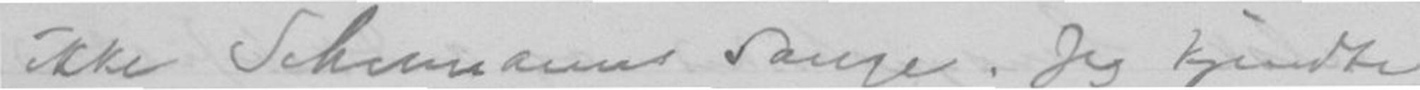ikke Schumanns Sange. Jeg kjendte
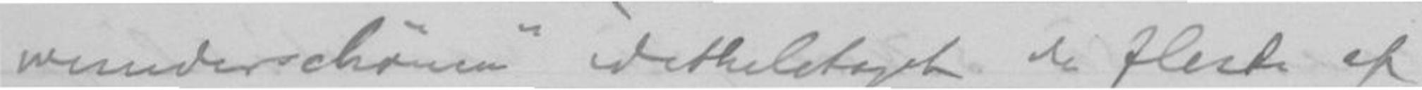wunderschønen" idetheletaget de fleste af
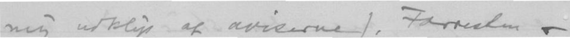mig udklip af aviserne). Forresten -
